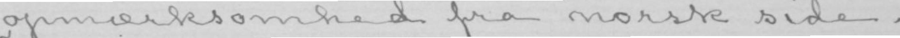opmærksomhed fra norsk side.
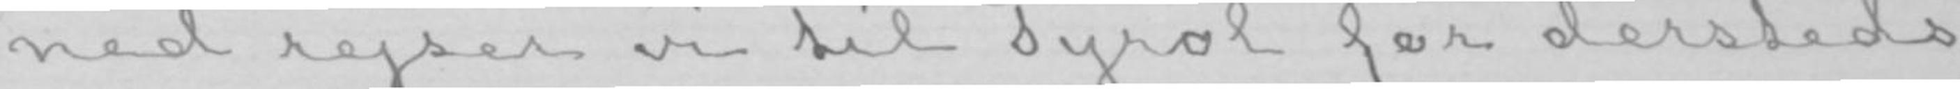ned rejser vi til Tyrol for dersteds
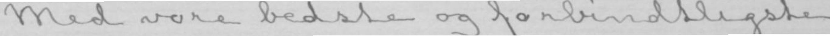Med vore bedste og forbindtligste
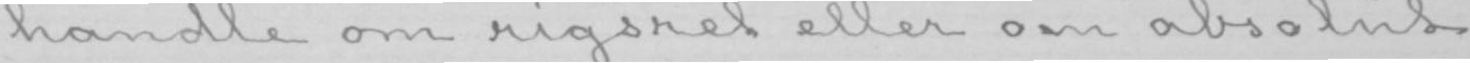handle om rigsret eller om absolut
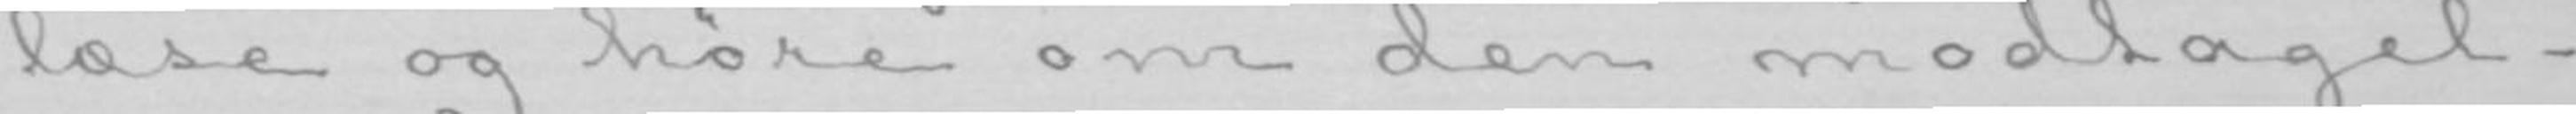læse og høre om den modtagel-
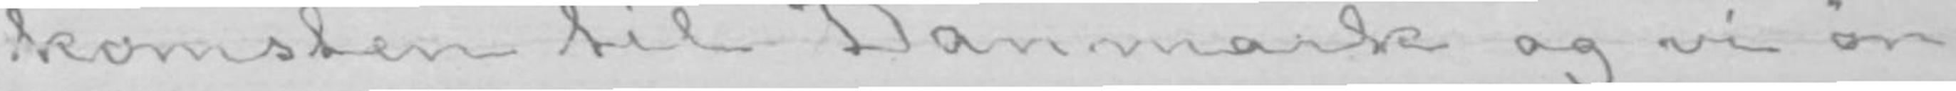komsten til Danmark og vi øn-
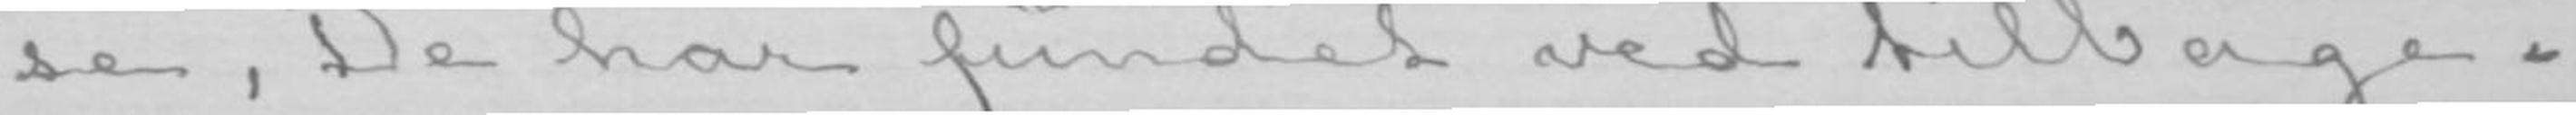se, De har fundet ved tilbage-
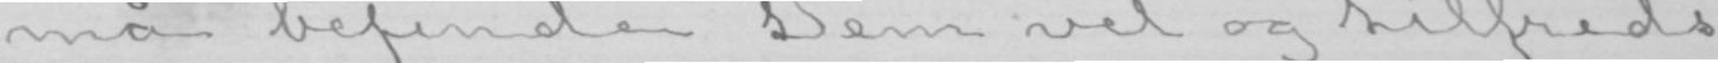må befinde Dem vel og tilfreds
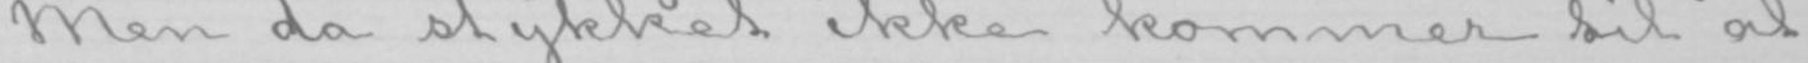Men da stykket ikke kommer til at
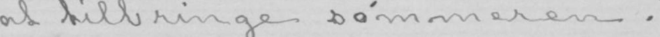at tilbringe sommeren.
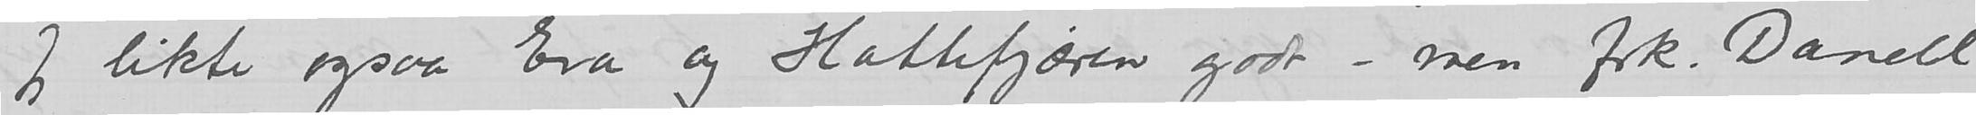Jeg likte ogsaa Eva og Hattefjæren godt - men frk. Danell
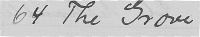64 The Grove
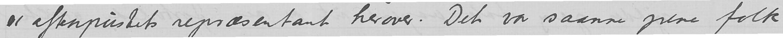er aftenpustets repræsentant herover. Det var saanne pene folk
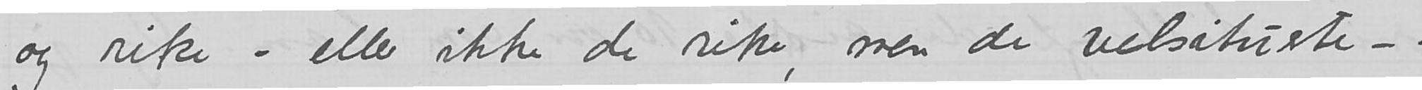og rike – eller ikke de rike, men de velsituerte -.
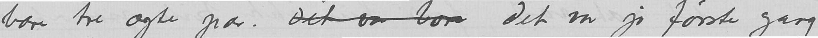bare tre ægte par. Det var bare Det var jo første gang
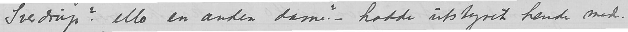Sverdrup? eller en anden dame? - hadde utstyret hende med.
